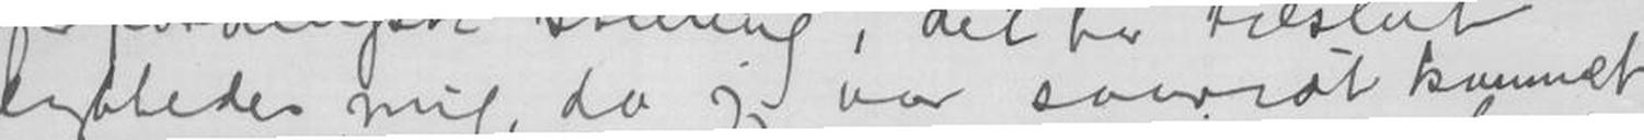lyktedes mig, da jeg var saavidt kommet
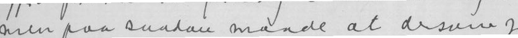men paa saadan maade at desværre jeg
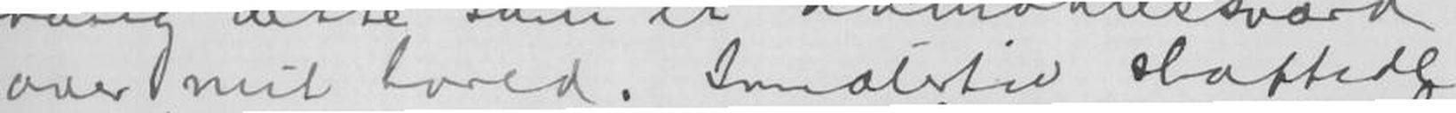over mit hoved. Imidlertid skaffede
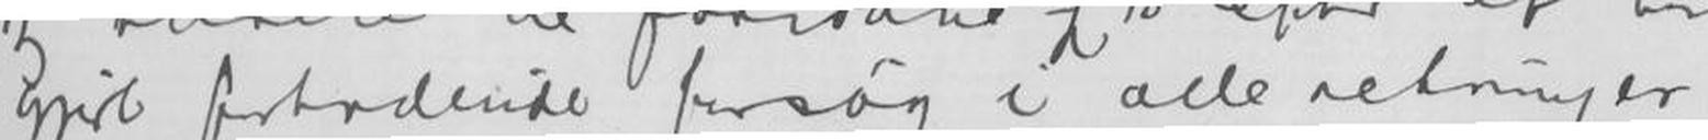gjort fortvilende forsøg i alle retninger,
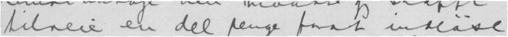tilveie en del penge forat indløse
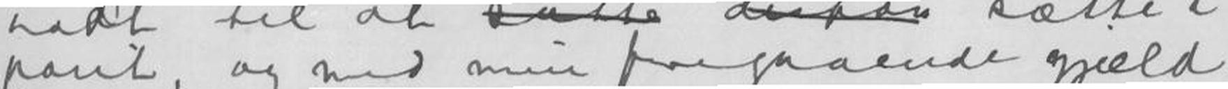pant, og med min foregaaende gjæld
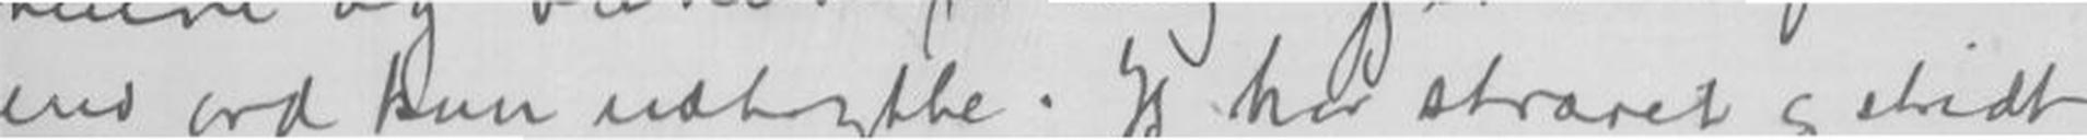end ord kan udtrykke. Jeg hat strævet og stridt
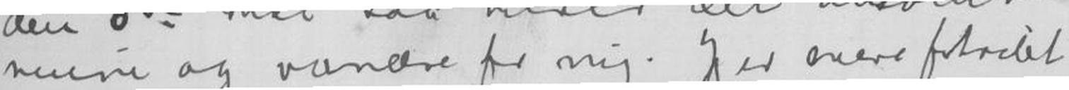og vanære for mig. Jeg er mere fortvilet
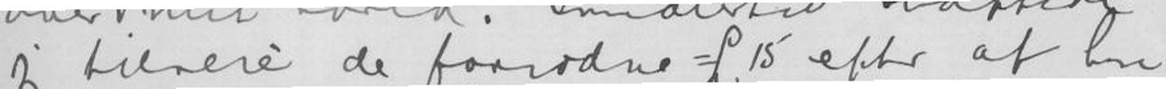jeg tilveie de fornødne =£15 efter at have
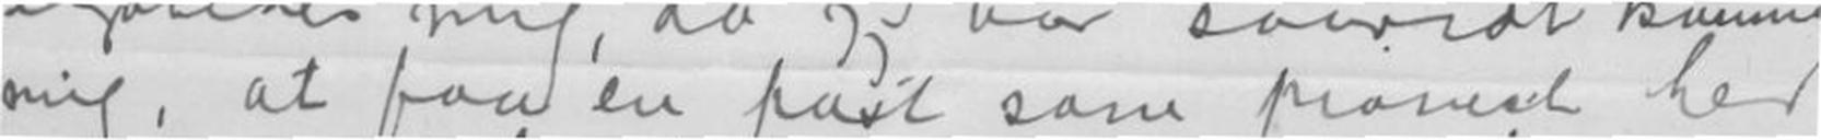mig, at faa en post som pianist her
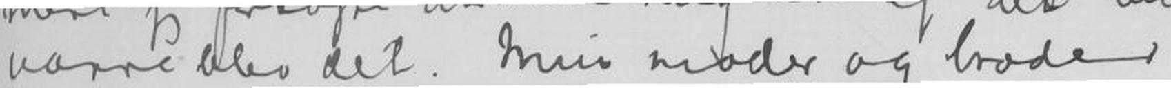værre blev det. Min moder og broder
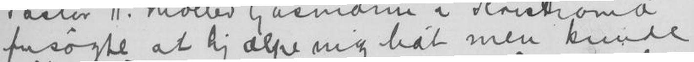forsøgte at hjelpe mig lidt men kunde
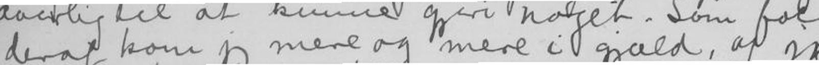deraf kom jeg mere og mere i gjæld, og jo
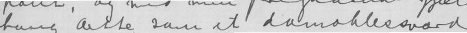hang dette som et damoklessværd
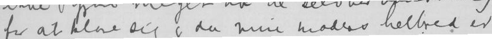for at klare sig og da min Moders helbred er
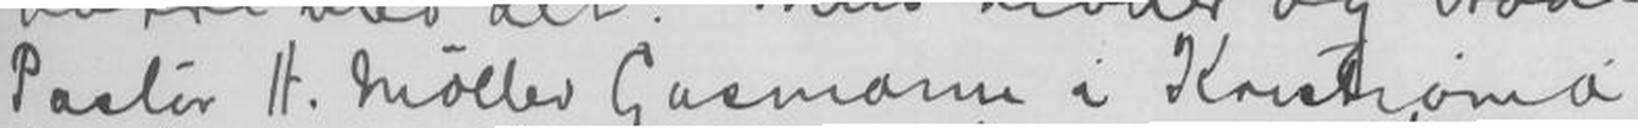Pastor H. Møller Gasmann i Kristiania
